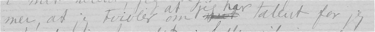mer, at jeg tvivler om mit talent for jeg
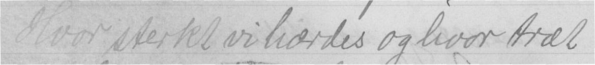Hvor sterkt vi hærdes og hvor træt
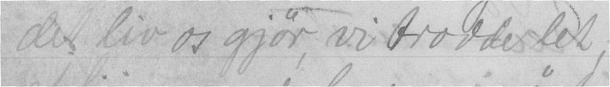det liv os gjør, vi trodde let
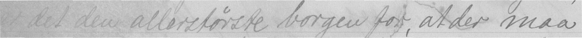er det den allerstørste borgen for, at der maa
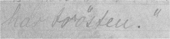har trøsten."
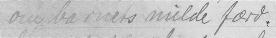om barnets milde færd.
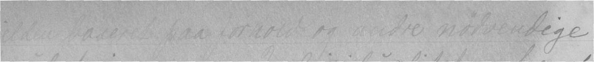sjelden baseret paa forhold og andre nødvendige
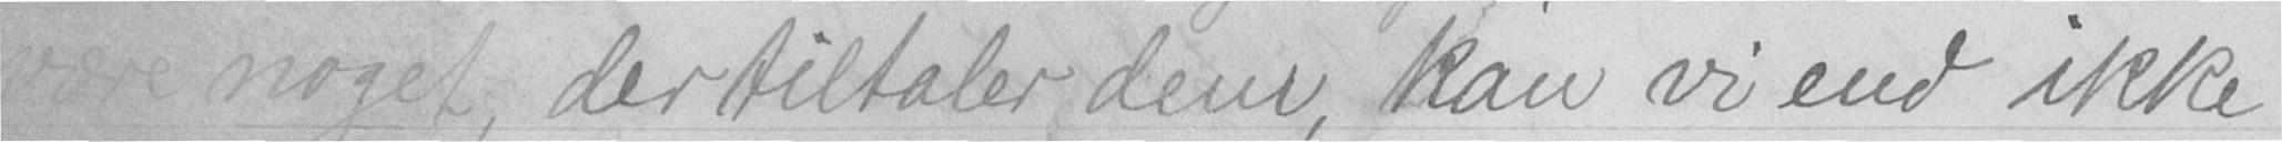være noget, der tiltaler dem, kan vi end ikke
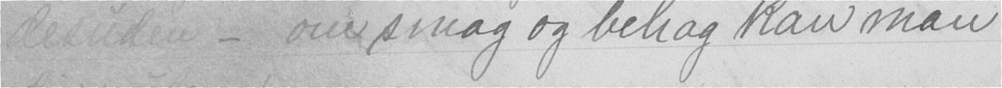desuden - om smag og behag kan man
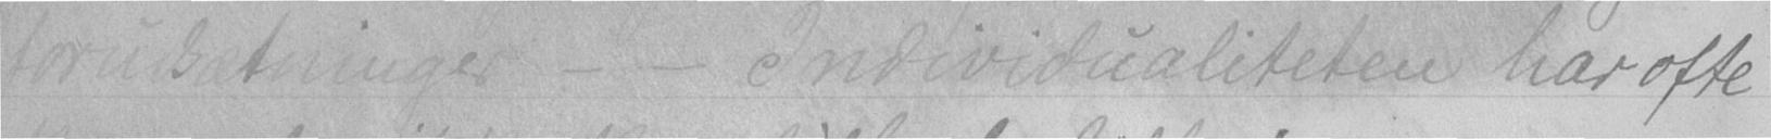forutsætninger - - Individualiteten har ofte
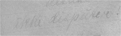ikke disputere!
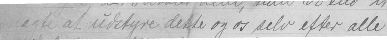magte at udstyre dette og os selv efter alle
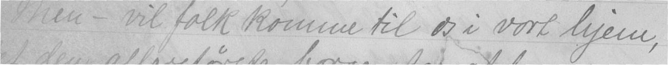Men - vil folk komme til os i vort hjem,
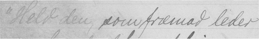"Held den, som fræmad leder
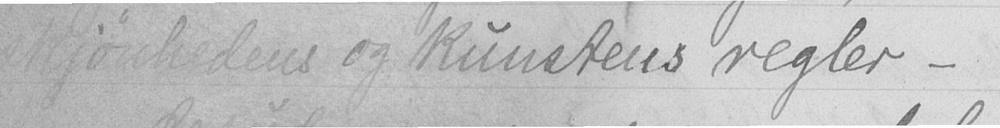skjønhedens og kunstens regler -
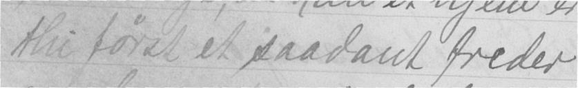thi først et saadant freder
